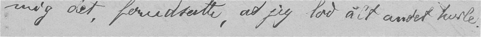mig det, forudsatte, at jeg lod alt andet hvile.
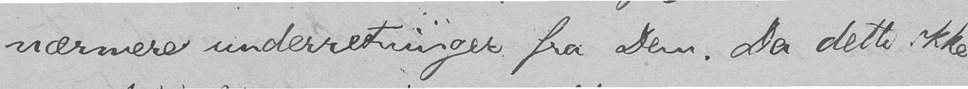nærmere underretninger fra Dem. Da dette ikke
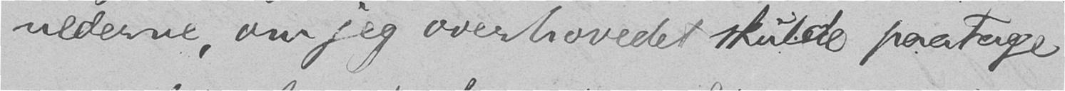nederne, om jeg overhovedet skulde paatage
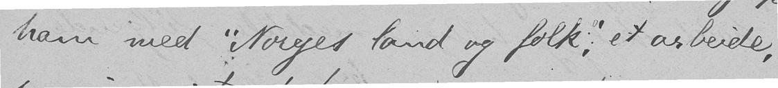ham med "Norges land og folk", et arbeide,
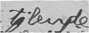tilende
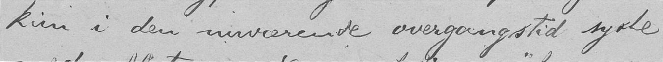kun i den nuværende overgangstid sysle
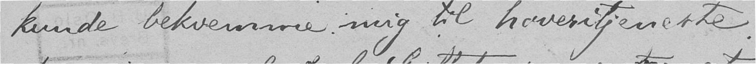kunde bekvemme mig til hoveritjeneste.
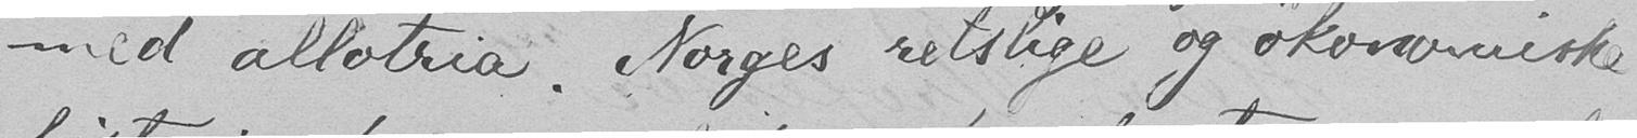med allotria. Norges retslige og økonomiske
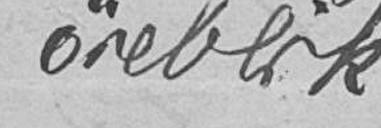øieblik
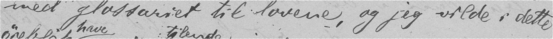med glossariet til lovene, og jeg vilde i dette
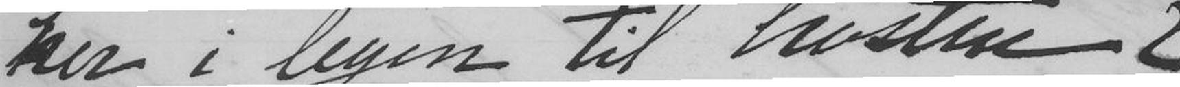her i byen til høsten?
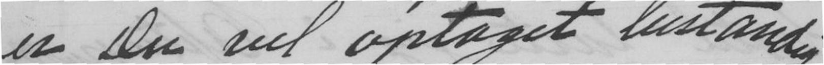er Du vel optaget bestandig,
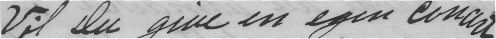Vil Du give en egen concert
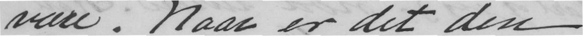være. Naar er det den
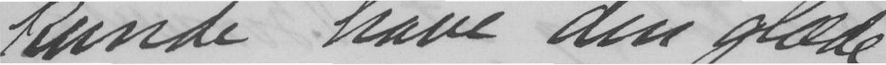kunde have den glæde
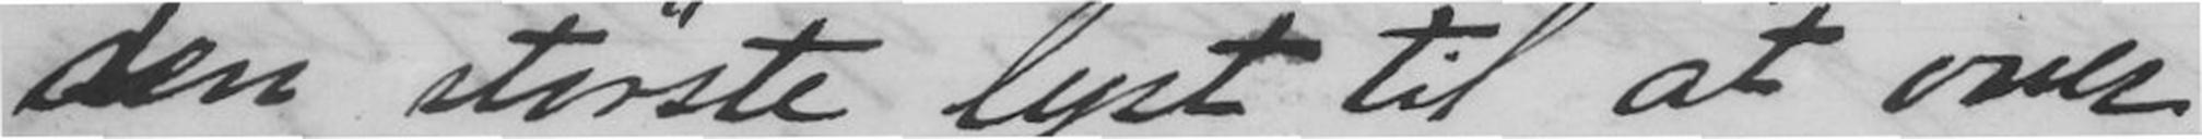den største lyst til at over-
